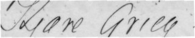Kjære Grieg
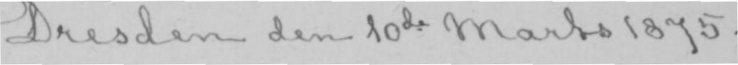Dresden den 10de Marts 1875.
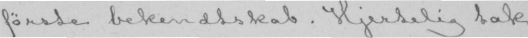første bekendtskab. Hjertelig tak
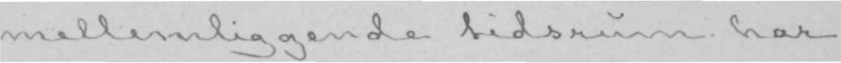mellemliggende tidsrum har
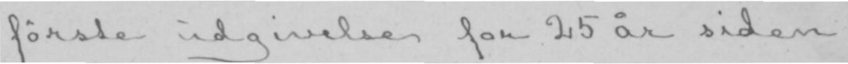første udgivelse for 25 år siden
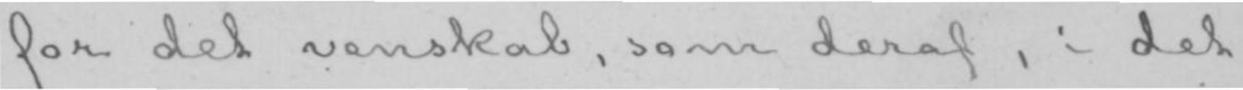for det venskab, som deraf, i det
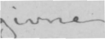givne
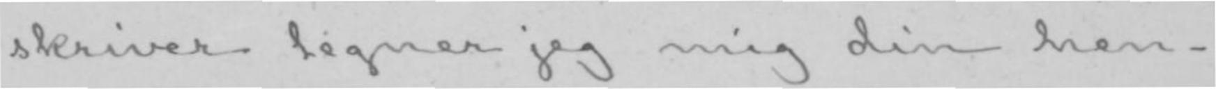skriver tegner jeg mig din hen-
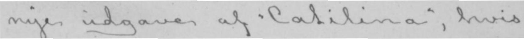nye udgave af «Catilina», hvis
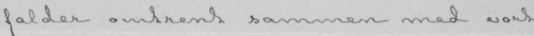falder omtrent sammen med vort
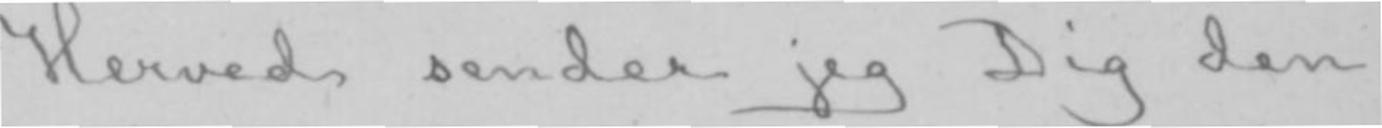Herved sender jeg Dig den
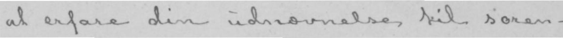at erfare din udnævnelse til soren-
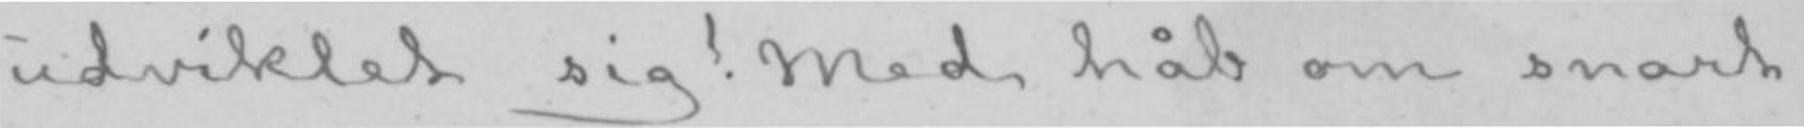udviklet sig! Med håb om snart
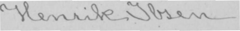Henrik Ibsen.
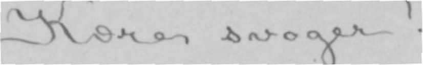Kære svoger!
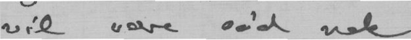vil være sød nok
